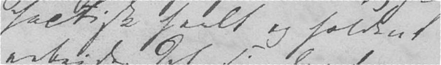factisk heelt og holdent
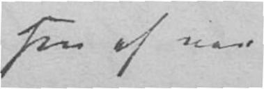sen af var
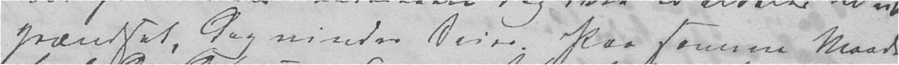grændset, dog vinder Seire. Paa samme Maade
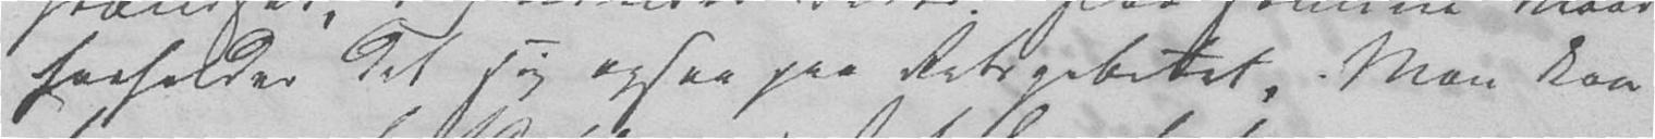forholder det sig ogsaa paa Retsgebetet. Man kan
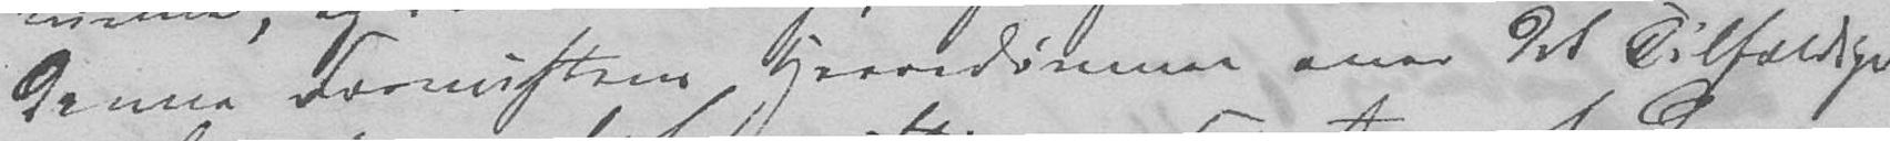denne Fornuftens Herredømme over det Tilfældige
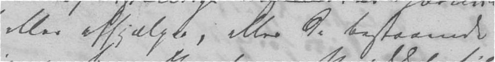eller afhjælpes, eller de bestaaende
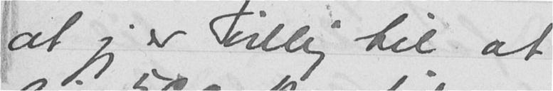at jeg er villig til at
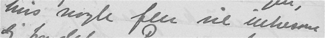hvis nogle fler vil intressere
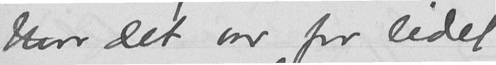hvor det var for lidet
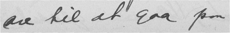sne til at gaa paa
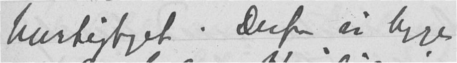hurtigtoget. Derfra vi begge
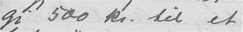gi 500 kr. til et
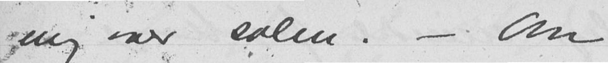mig over solen. - Om
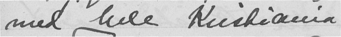med hele Kristiania
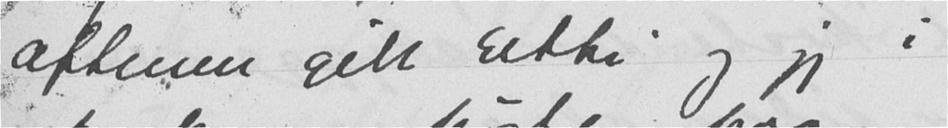aftenen gik Etti og jeg i
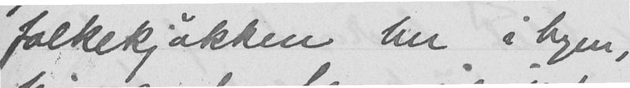folkekjøkken her i byen,
